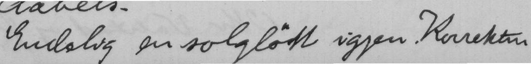Endelig en solgløtt igjen. Korrekur
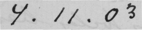4.11.03
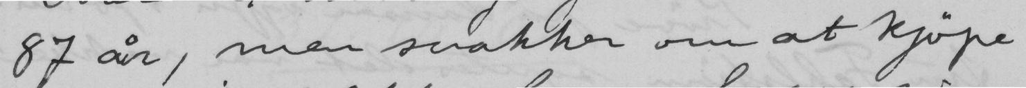87 år, men snakker om at kjøpe
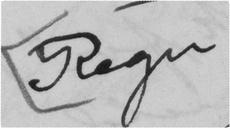Regn
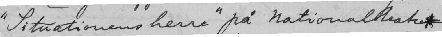"Situationens herre" på Nationalteateret
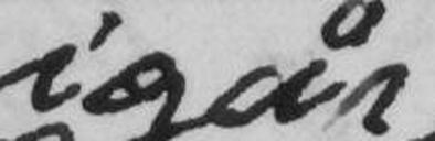igår
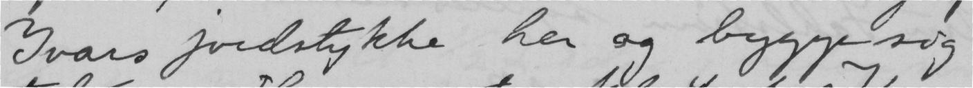Ivars jordstykke her og bygge sig
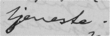tjeneste.
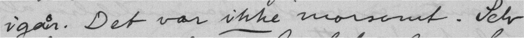igår. Det var ikke morsomt. Selv
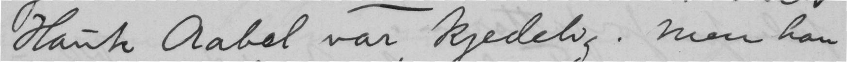Hauk Aabel var kjedelig. Men han
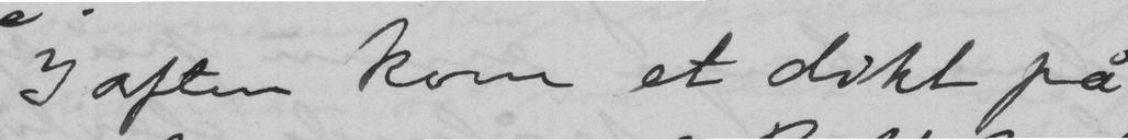I aften kom et dikt på
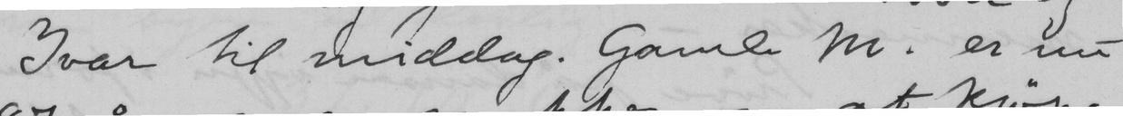Ivar til middag. Gamle M. er nu
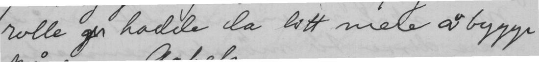rolle  hadde da litt mere å bygge
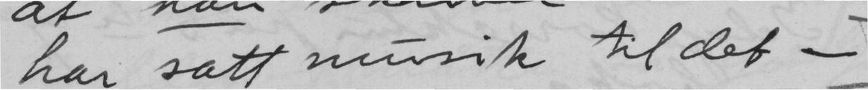har satt musik til det —
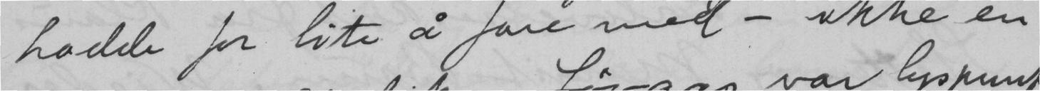hadde for lite å fare med - ikke én
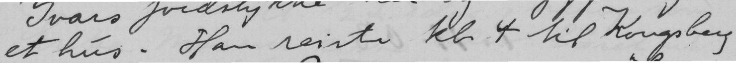et hus. Han reiste kl. 7 til Kongsberg
